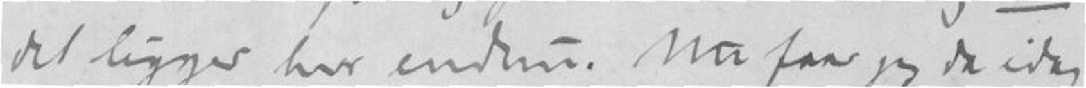det ligger her endnu. Nu faar jeg da idag
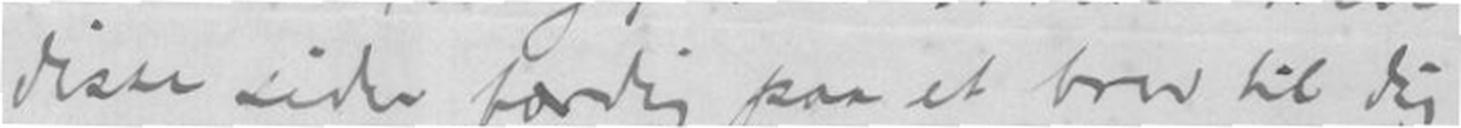disse sider ferdig paa et brev til dig
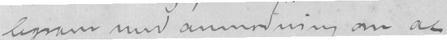legram med anmodning om at
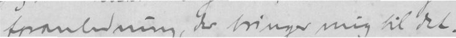foranledning, der bringer mig til det.
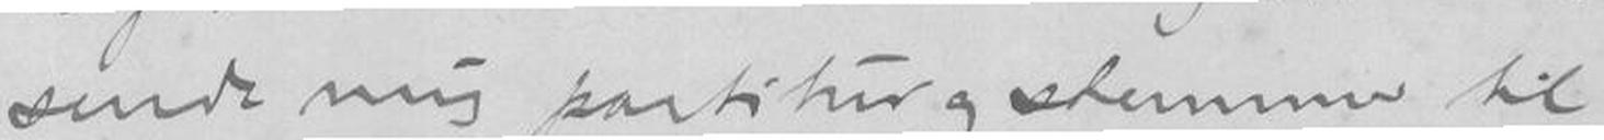sende mig partitur og stemmer til
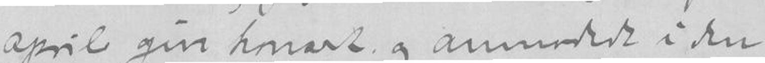april give konsert og anmodedt i den
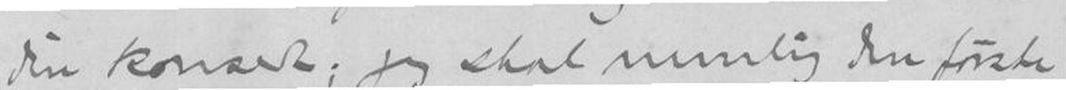din konsert; jeg skal nemlig den første
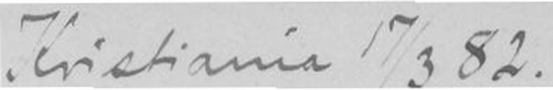Kristiania 17/3 82
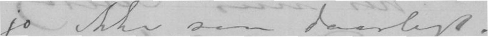jo ikke saa daarligt.
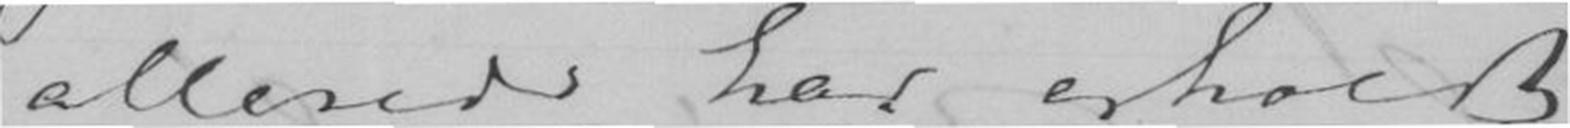allerede har erholdt
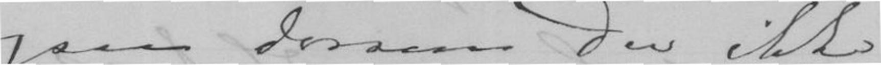ogsaa dersom Du ikke
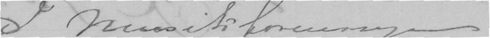I Musikforeningen
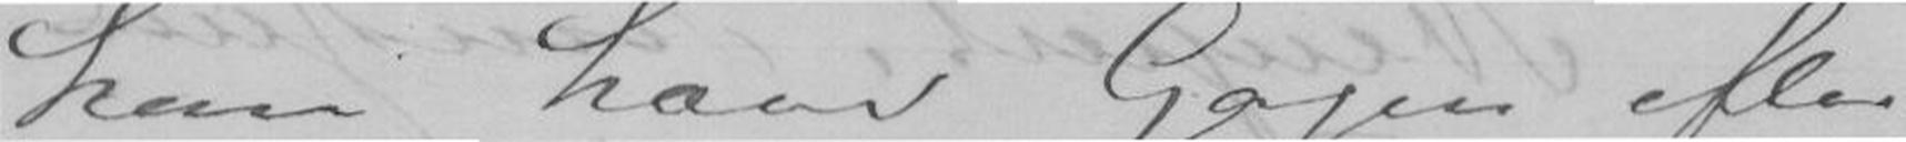kan have Gagen efter
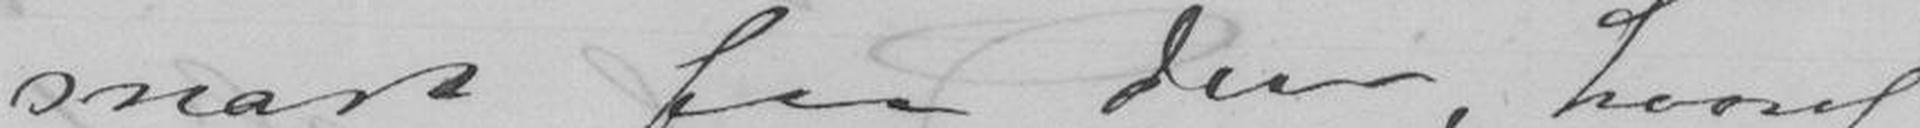snart faa den, hvoraf
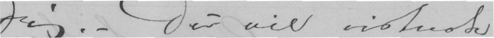Dig. - Du vil vistnok
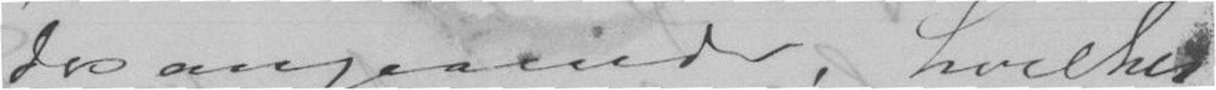des angaaende, hvilket
